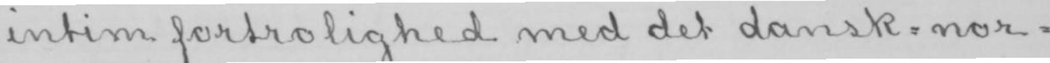intim fortrolighed med det dansk-nor-
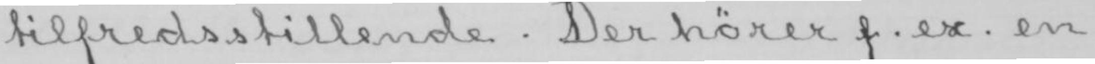tilfredsstillende. Der hører f. ex. en
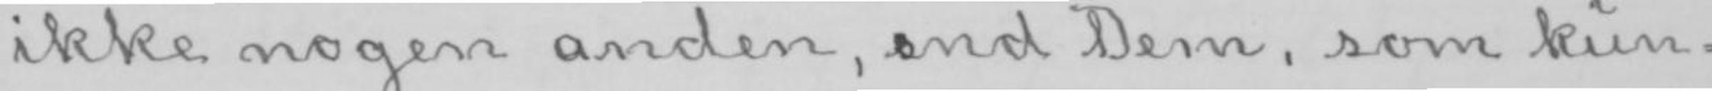ikke nogen anden, send Dem, som kun-
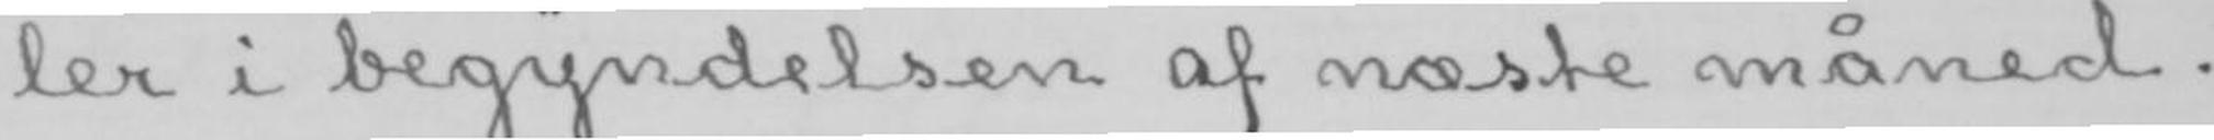ler i begyndelsen af næste måned.
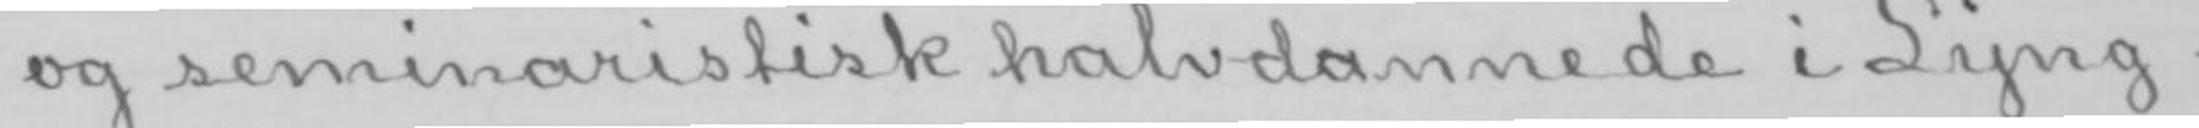og seminaristisk halvdannede i Lyng-
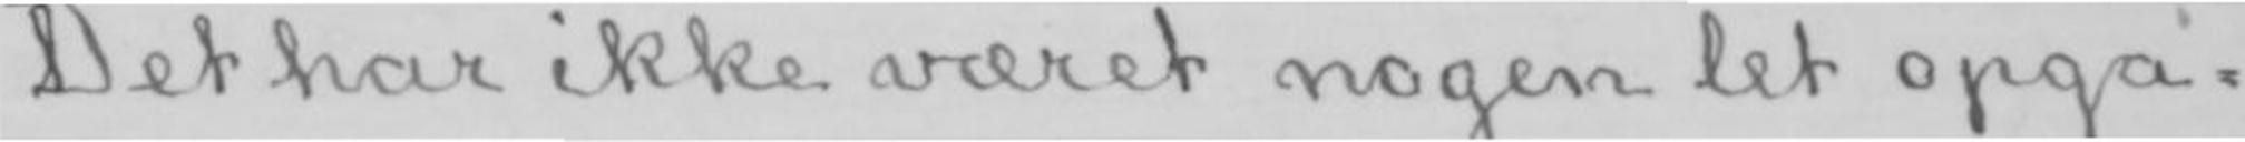Det har ikke været nogen let opga-
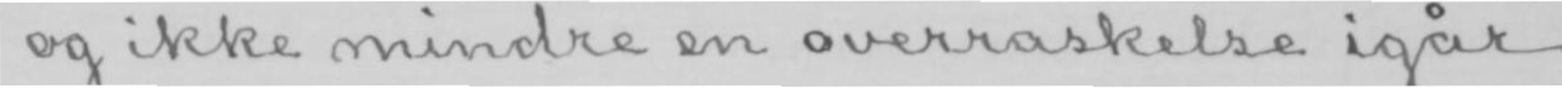og ikke mindre en overraskelse igår
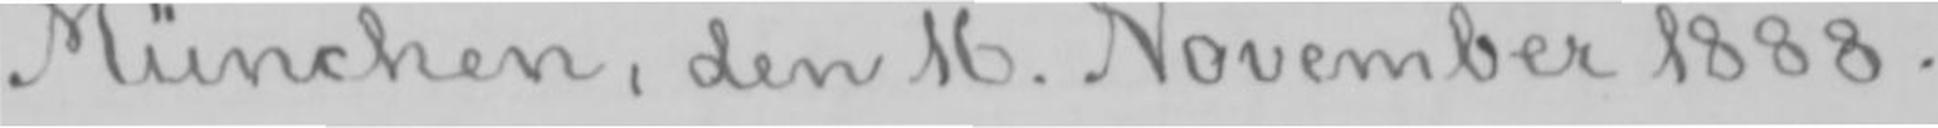München, den 16. November 1888.
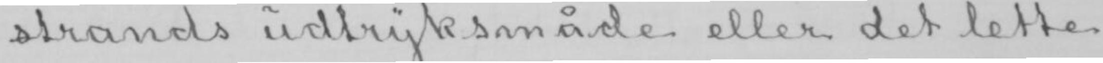strands udtryksmåde eller det lette
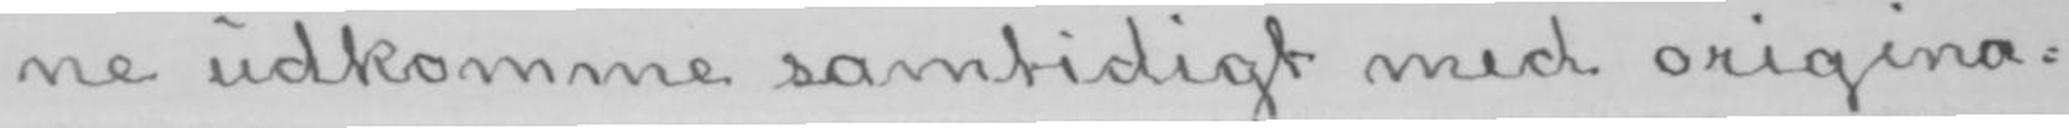ne udkomme samtidigt med origina-
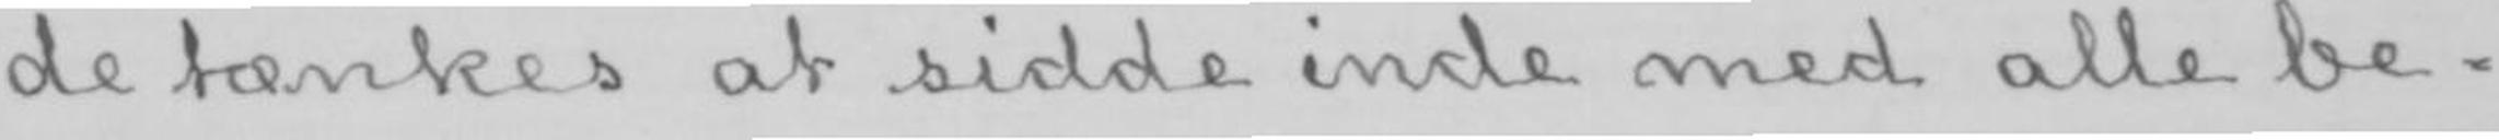de tænkes at sidde inde med alle be-
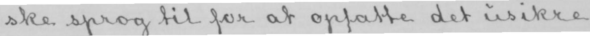ske sprog til for at opfatte det usikre
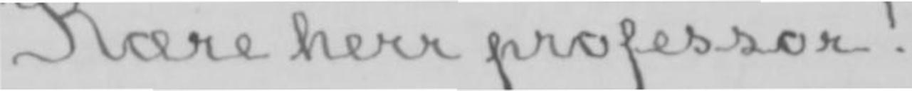Kære herr professor!
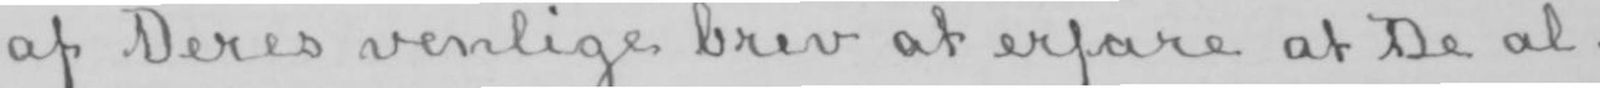af Deres venlige brev at erfare at De al-
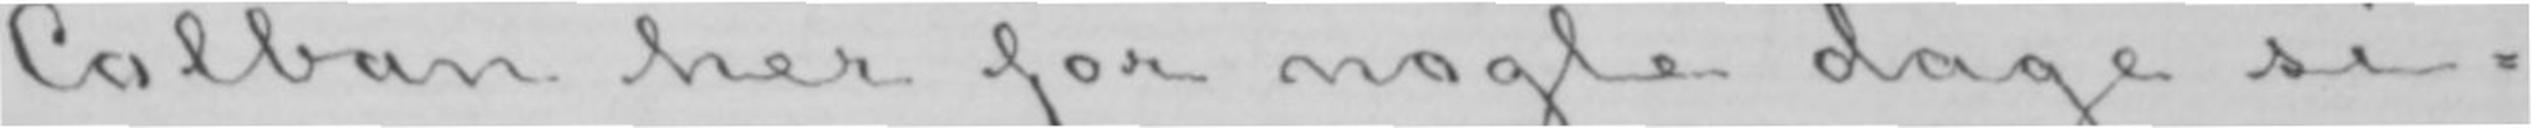Colban her for nogle dage si-
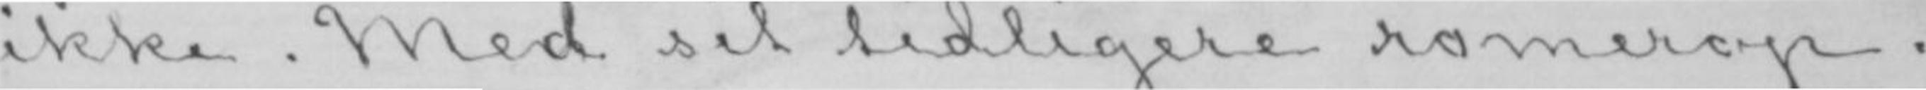ikke. Med sit tidligere romerop-
